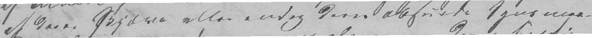af deres skjæve eller endog deres absurde Synsmaa-
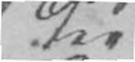Der
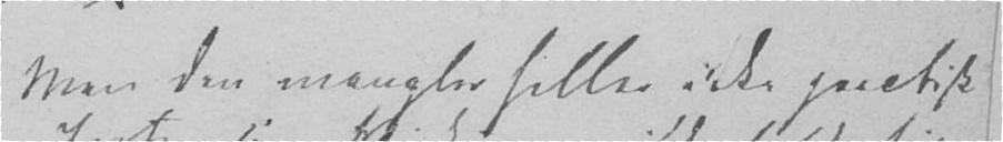Men den mangler heller ikke practisk
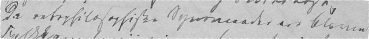De retsphilosophiske Synsmaader ere blevne
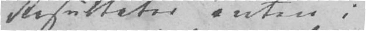Resultater enten i
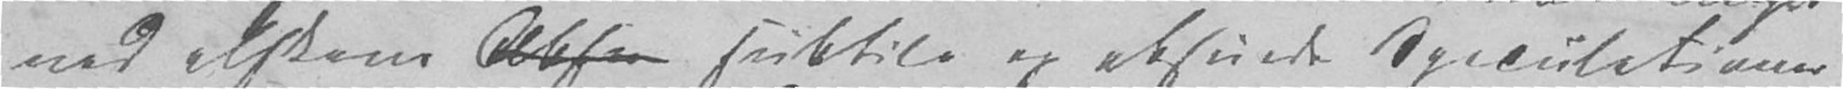ved alskens subtile og absurde Speculationer
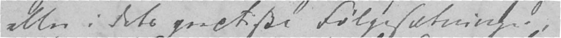eller i dets practiske Følgesætninger,
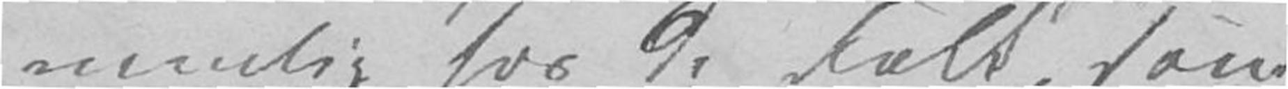nemlig hos de Folk, som
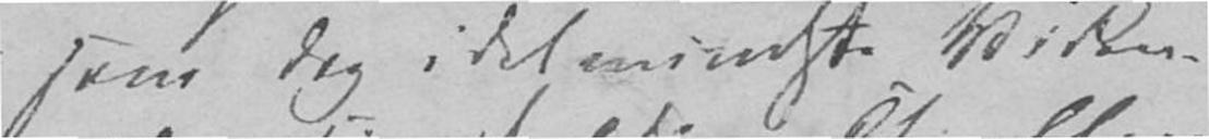som dog idetmindste Viden-
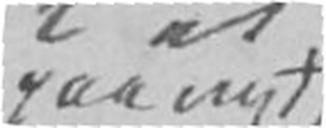paa nyt
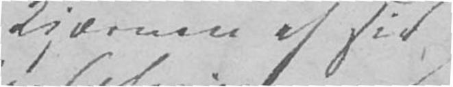Kjærnen af sit
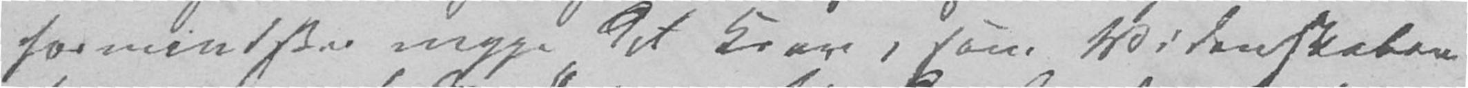formindskes neppe det Krav, som Videnskaben
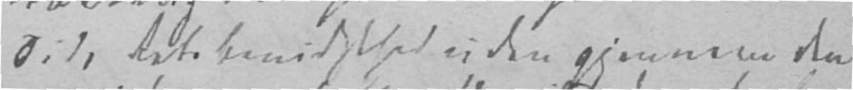Tids Retsbevidsthed uden gjennem den
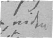, viden-
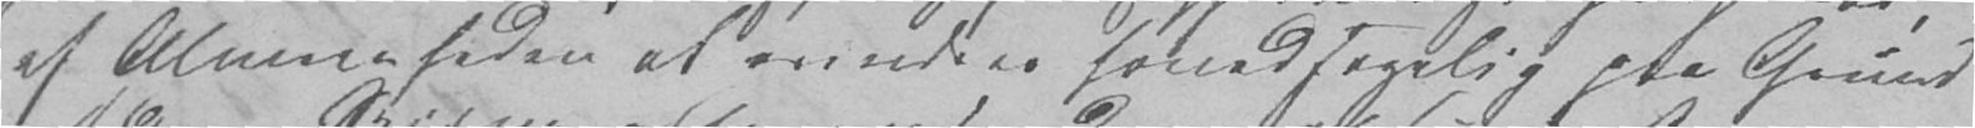af Almeenheden at anvendes hovedsagelig paa Grund
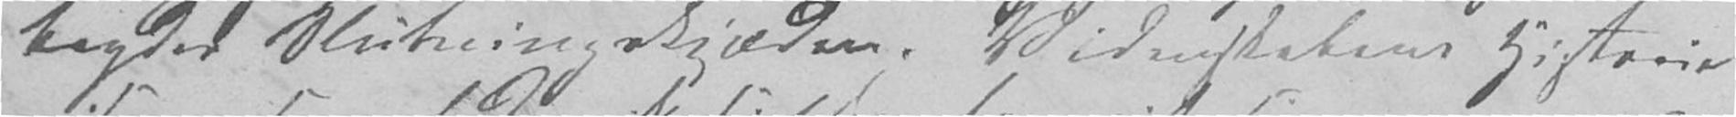bryder Slutningskjæden. Videnskabens Historie
